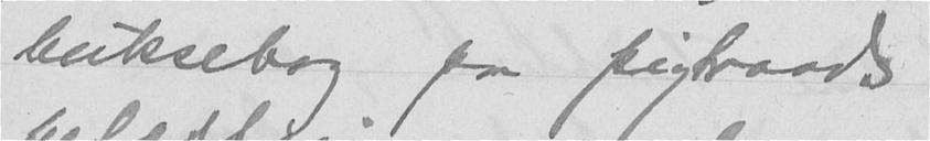buksebag paa pigtraads
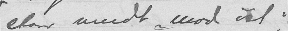staar vendt mod øst,
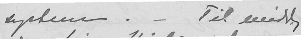system. - Til middag
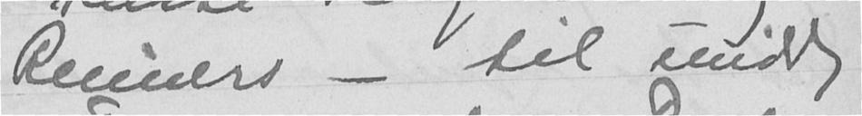Reimers - til middag
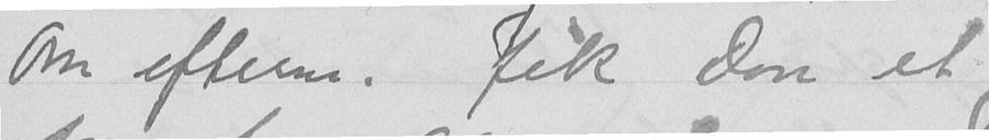Om efterm. fik Don et
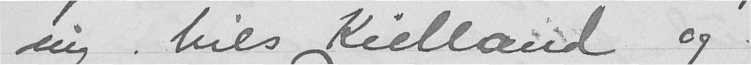mig, Nils Kielland og
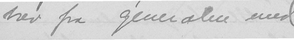brev fra generalen med
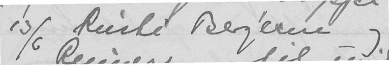13/6 Reiste Berg'erne og
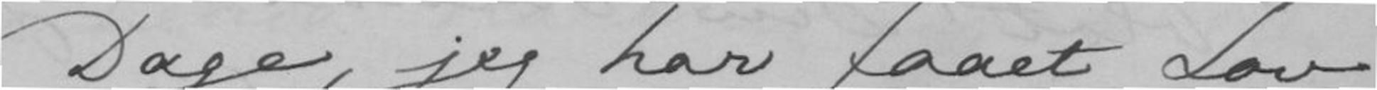Dage, jeg har faaet Lov
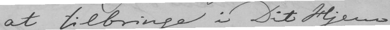at tilbringe i Dit Hjem
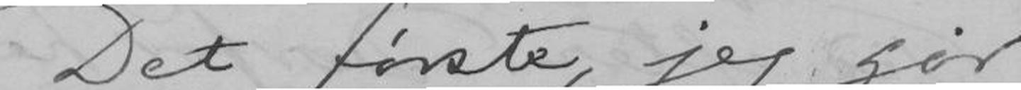Det første, jeg gør
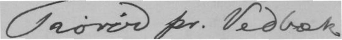Trørød pr. Vedbæk.
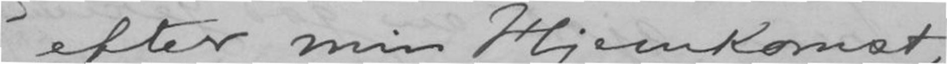efter min Hjemkomst,
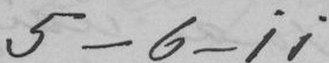5-6-11
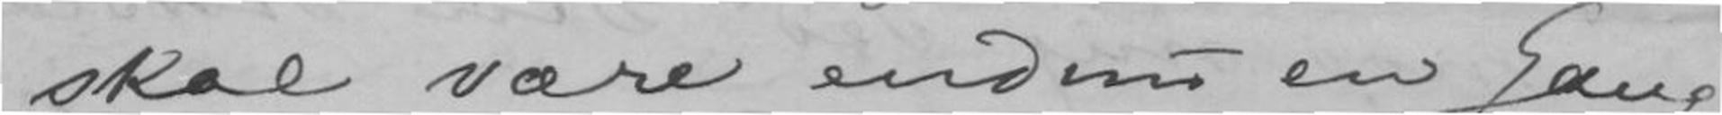skal være endnu en Gang
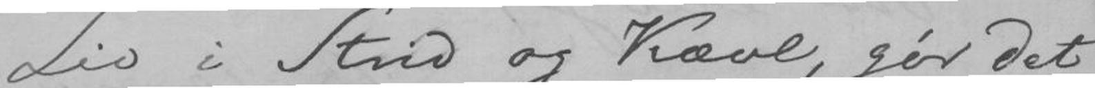Liv i Strid og Kævl, gør det
